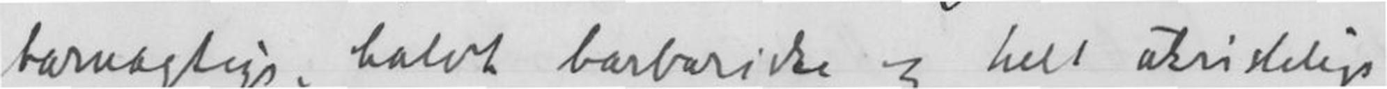barnagtige, halvt barbariske og helt ukristelige
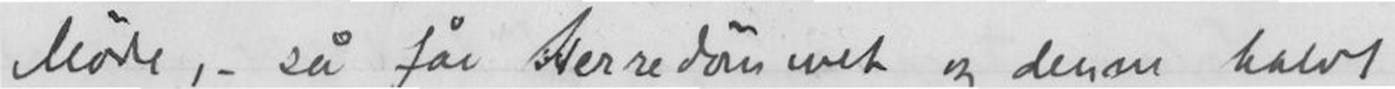Møde, - så får herredømmet og denne halvt
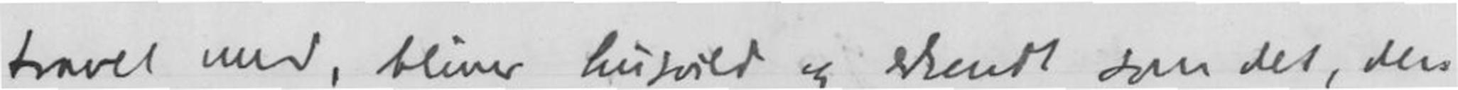travel med, bliver husvild og erkendt som det, dere
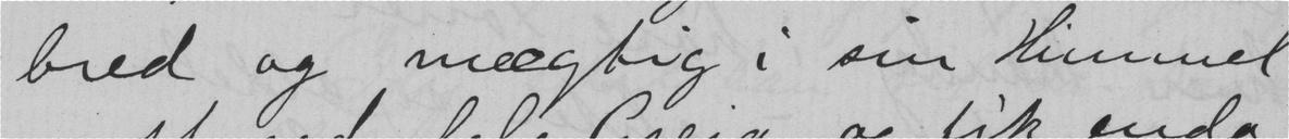bred og mægtig i sin Himmel
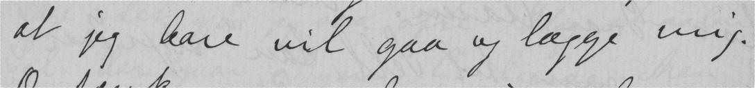at jeg bare vil gaa og lægge mig.
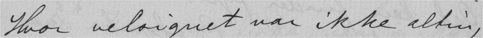Hvor velsignet var ikke alting
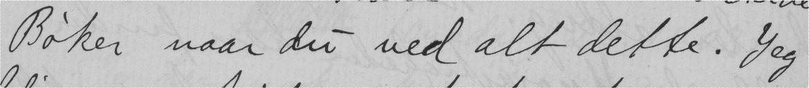Bøker naar du ved alt dette. Jeg
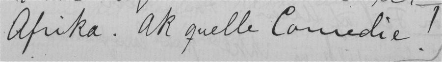Afrika. Ak quelle Comedie!
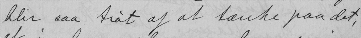blir saa trøt af at tænke paa det,
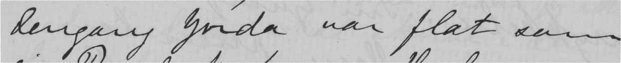dengang jorda var flat som
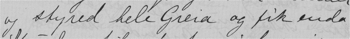og styred hele Greia og fik enda
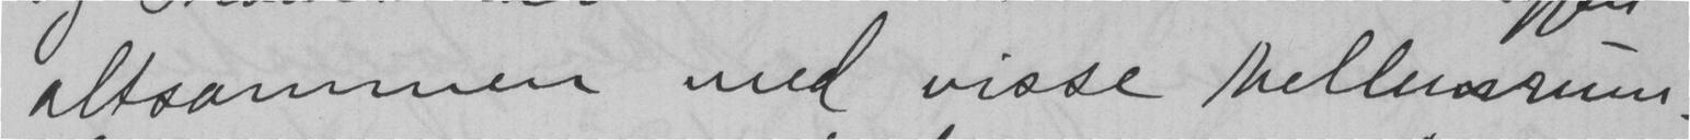altsammen med visse mellumrum.
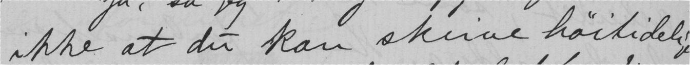ikke at du kan skrive høitidelige
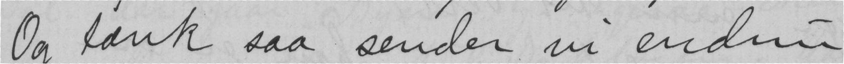Og tænk saa sender vi endnu
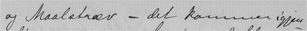og Maalstræv - det kommer igjen
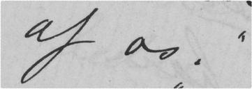af os."
